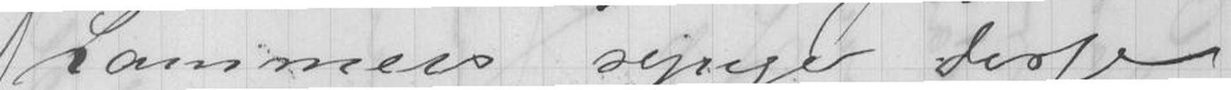Lammers synge desse
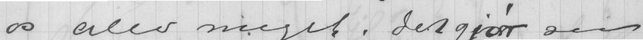os alle meget. Det gjør saa
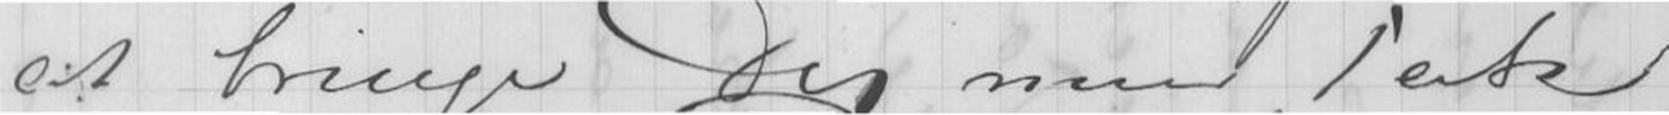at bringe Dig med Tak
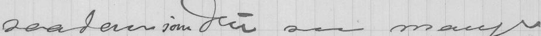saadan som Du saa mange
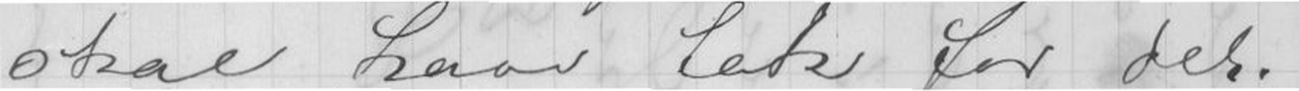skal have tak for det.
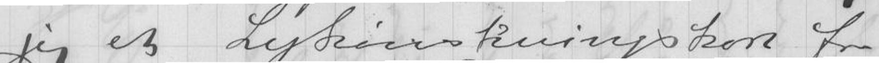jeg et Lykønskningskort fra
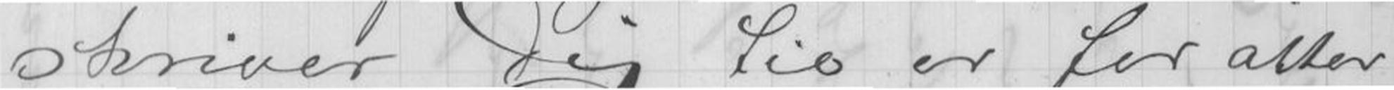skriver Dig til er for atter
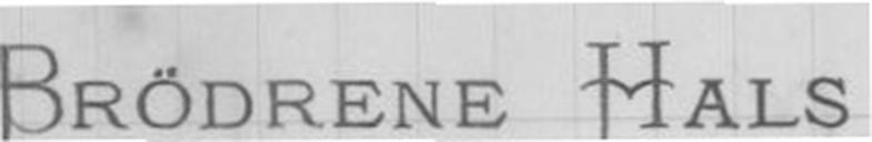Brødrene Hals
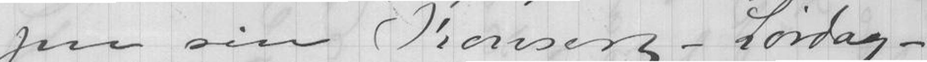paa sin Konsert - Lørdag -
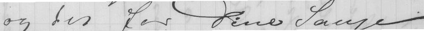og det for Dine Sange
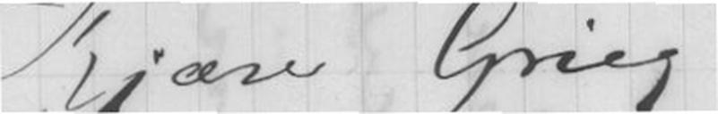Kjære Grieg
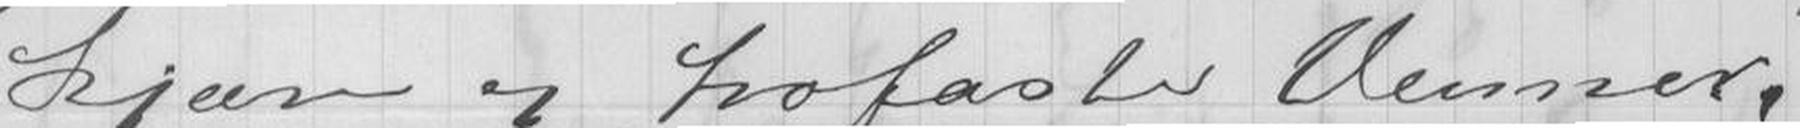kjære og trofaste Venner.
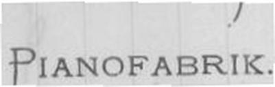Pianofabrik.
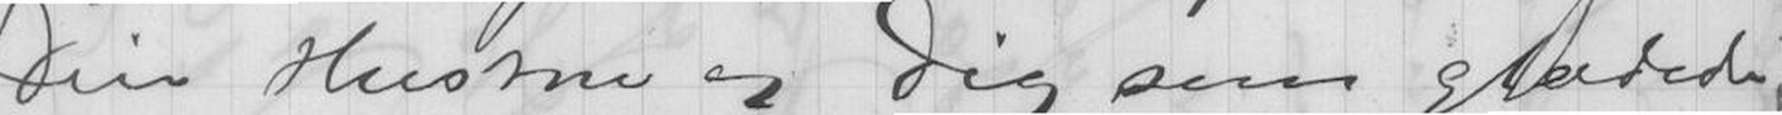Din Hustru og Dig som glædede
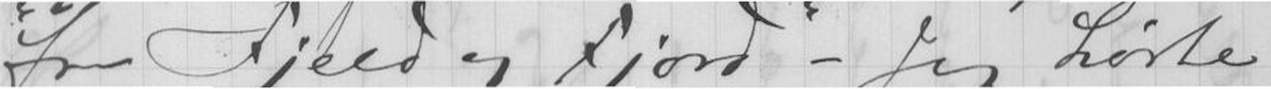"fra Fjeld og Fjord" - Jeg hørte
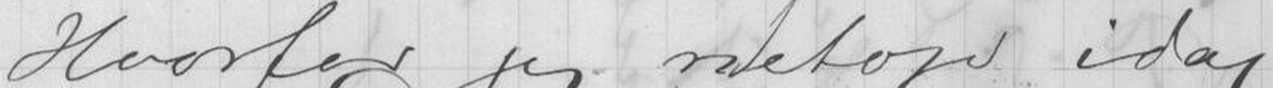Hvorfor jeg netop idag
